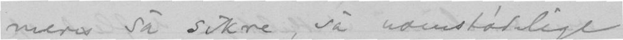meres så sikre, så uanstødelige
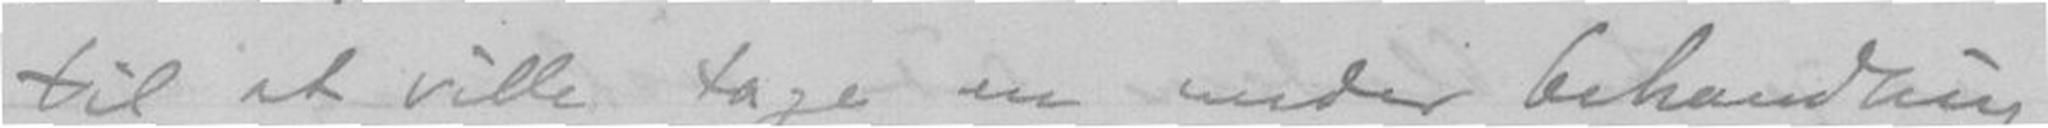til at ville tage en under behandling.
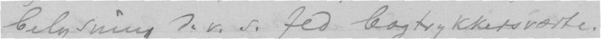belysning d.v.s. fed bogtrykkersværte.
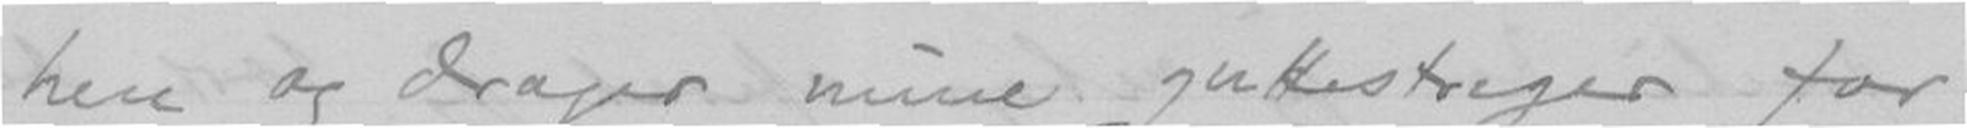hen og drager mine guttestreger for
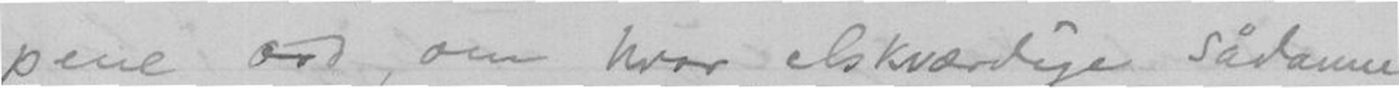pene ord, om hvor elskværdige sådanne
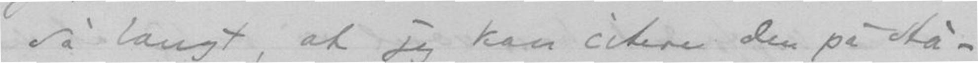så langt, at jeg kan citere den på stå-
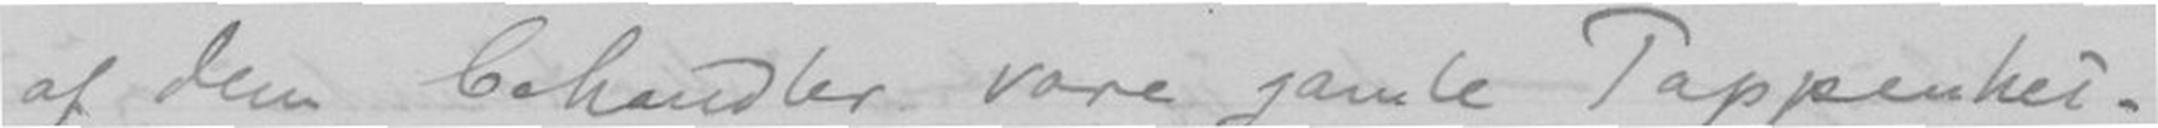af den behandler vore gamle Tappenhei-
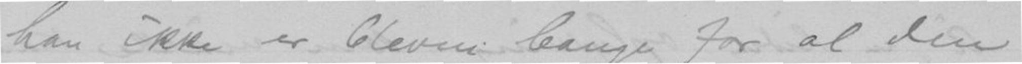han ikke er blivet bange for al den
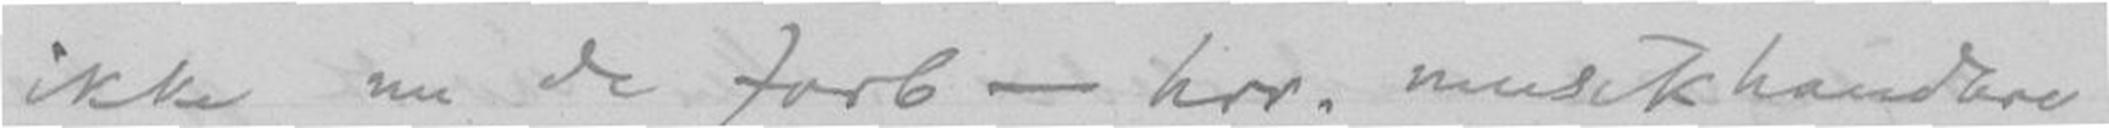ikke nu de forb - hrr. musikhandlere
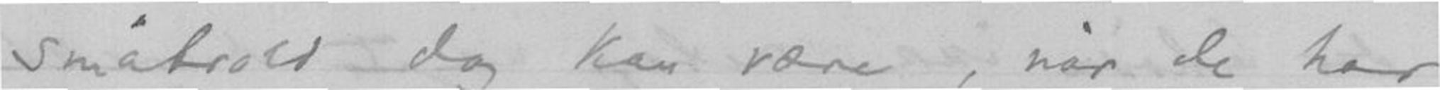småtrold dog kan være, når de har
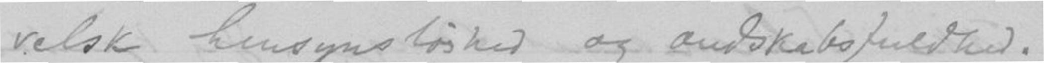velsk hensynsløshed og ondskabsfuldhed.
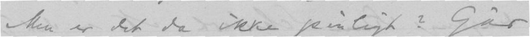Men er det da ikke pinligt? Går
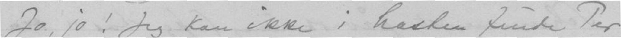Jo, jo! Jeg kan ikke i hasten finde Per
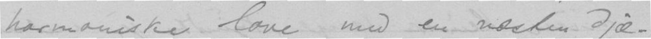hamoniske love med en næsten djæ-
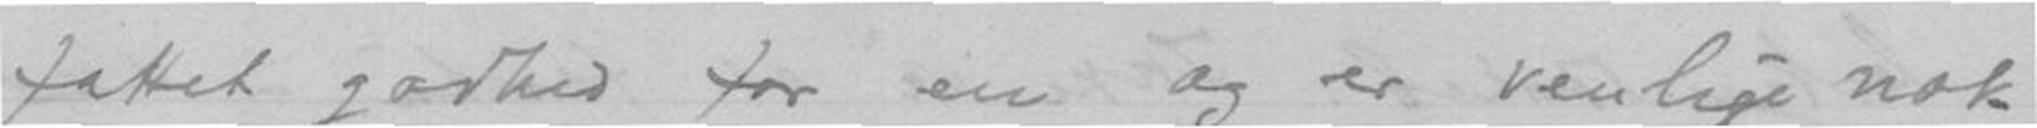fattet godhed for en og er venlige nok
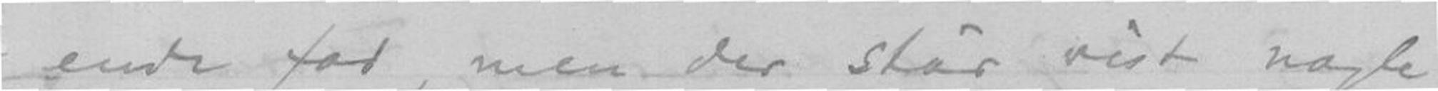ende fod, men der står vist nogle
